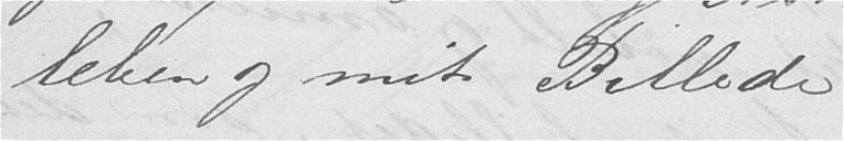leben og mit Billede.
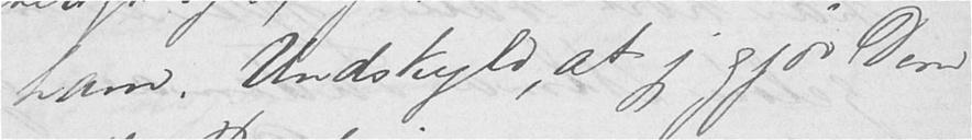ham. Undskyld, at jeg gjør Dem
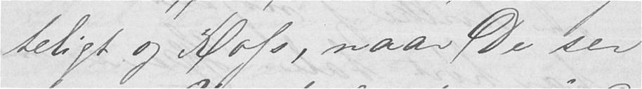teligt og Hofs, naar De ser
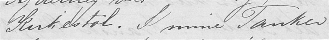Kirkestol. I mine Tanker
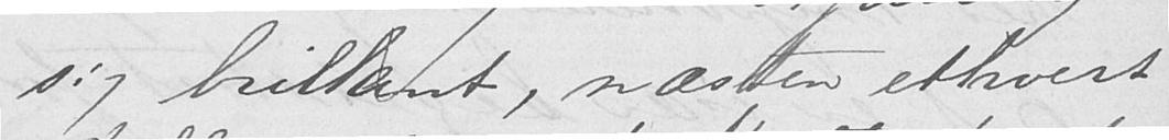sig brillant, næsten ethvert
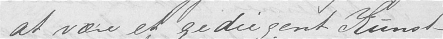at være et gediegent Kunst-
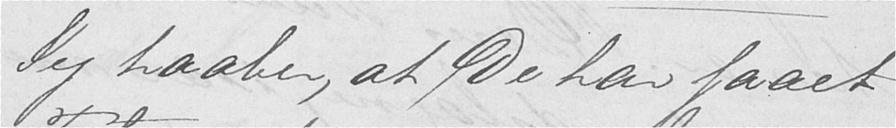Jeg haaber, at De har faaet
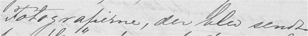Fotografierne, der blev sendt
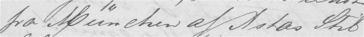fra München af Astas Stil-
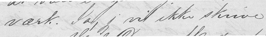værk. Ja, jeg vil ikke skrive
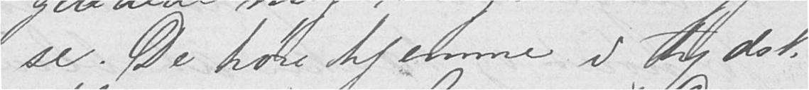se. De høre hjemme i tydsk
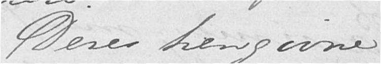Deres hengivne
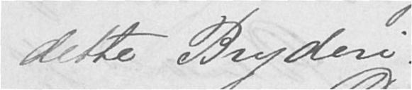dette Bryderi.
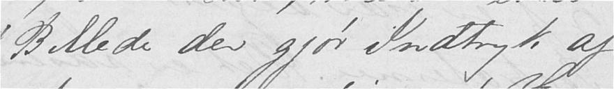Billede der gjør Indtryk af
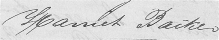Harriet Backer -
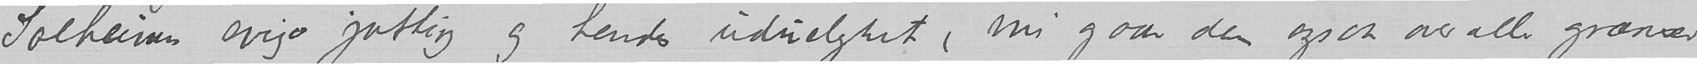Solheims evige jatting og hendes uduelighet, (nu gaar den ogsaa over alle grænser
